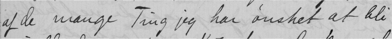af de mange Ting jeg har ønsket at bli
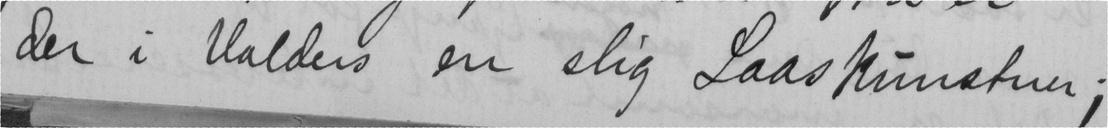der i Valders en slig Laaskunstner;
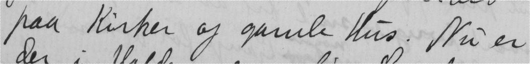paa Kirker og gamle Hus. Nu er
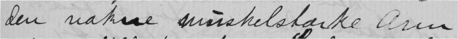den vakre muskelstærke arm
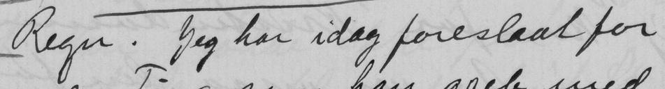Regn. Jeg har idag foreslaat for
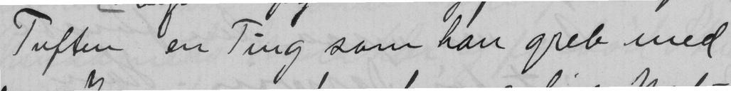Tuften en Ting som han greb med
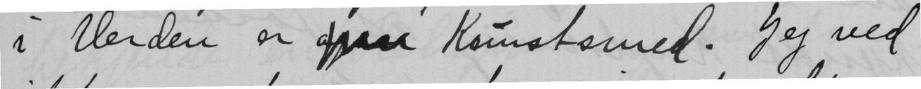i verden er  Kunstsmed. Jeg ved
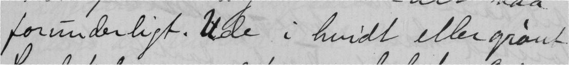forunderligt. Ude i hvidt eller grønt
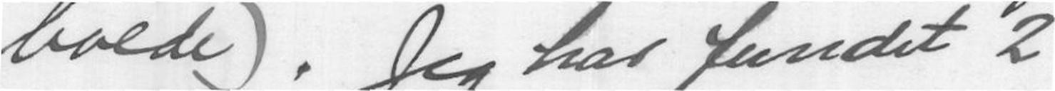boede). Jeg har fundet 2
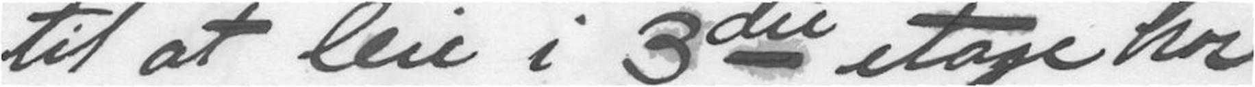til at leie i 3die etage hos
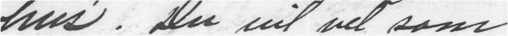hus. Du vil vel som
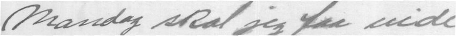Mandag skal jeg faa vide
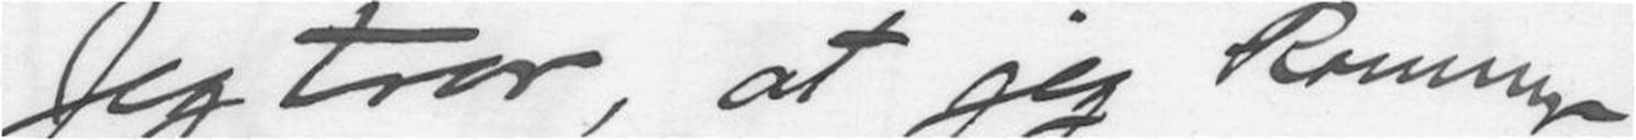Jeg tror, at jeg kommer
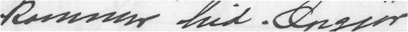kommer hid Opgjør
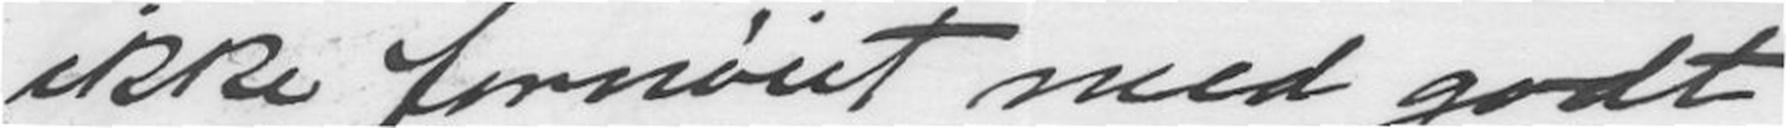ikke fornøiet med godt
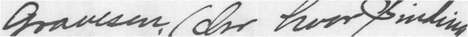Gravesen, (der hvor Sinding
